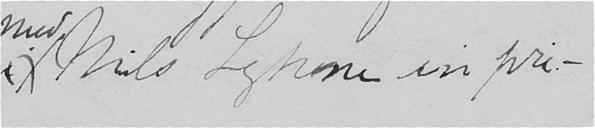i Nils Lyhne in pri-
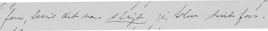fen, hvis det var sligt jeg blev sint for.
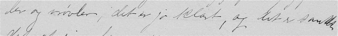ler og vrøvler; det er jo klart, og det er sandt
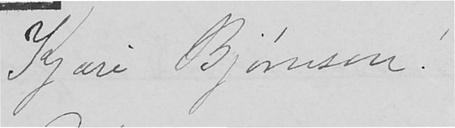Kjære Bjørnson!
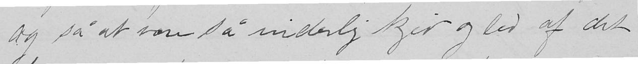og så at være så inderlig kjed og lei af det
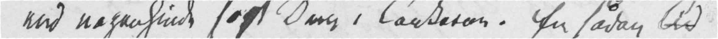end nogensinde søgt Dem i Tankerne. En sådan Tid
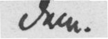dem.
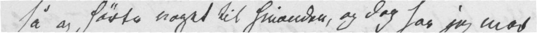så og hørte noget til hinanden, og dog har jeg mer
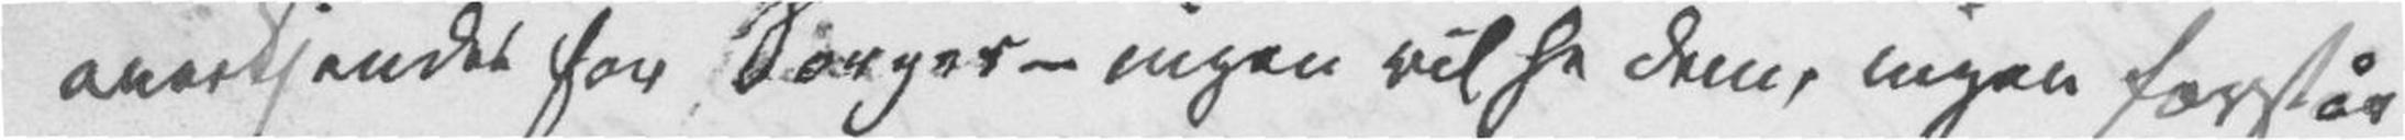anerkjendes for Sorger – ingen vil se dem, ingen forstår
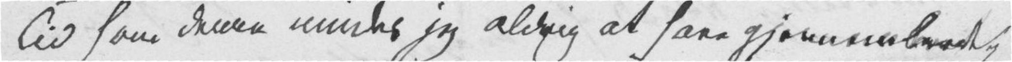Tid som denne mindes jeg aldrig at have gjennemlevet.
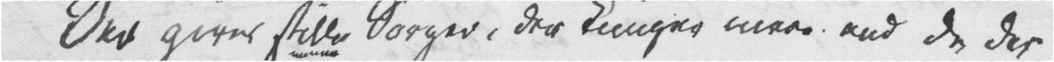Der gives stille Sorger, der knuger mere end de der
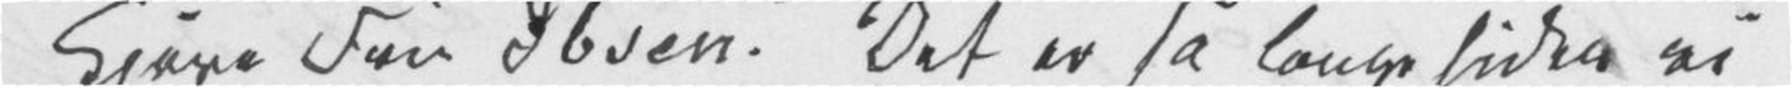Kjere Fru Ibsen! Det er så længe siden vi
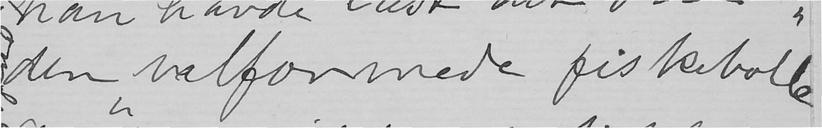den "velformede fiskebolle"
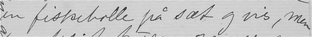en fiskebolle på sæt og vis, men
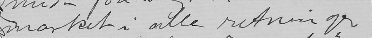mærket i alle retninger
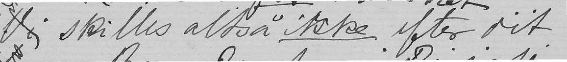Vi skilles altså ikke efter dit
